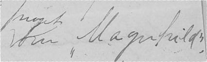om "Magnhild".
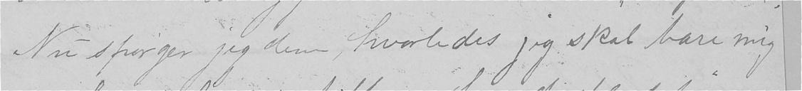Nu spørger jeg dem, hvorledes jeg skal bære mig
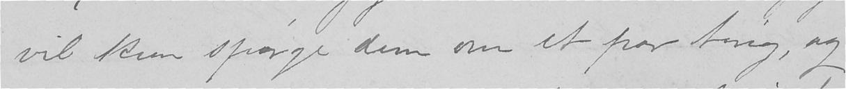vil kun spørge dem om et par ting, og
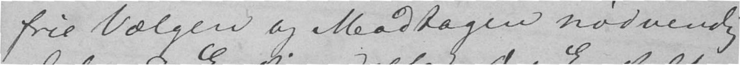frie Vælgen og Modtagen nødvendig
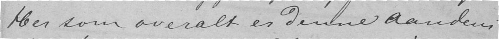Men som overalt er denne Aandens
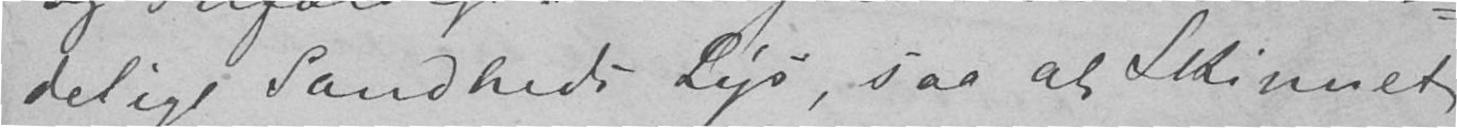delige Sandheds Lys, saa at Skinnet
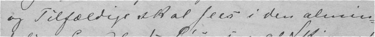og Tilfældige skal sees i den almin-
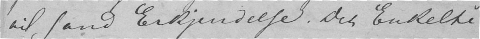til sand Erkjendelse. Det Enkelte
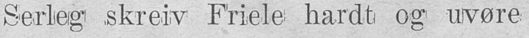Serleg skreiv Friele hardt og uvøre
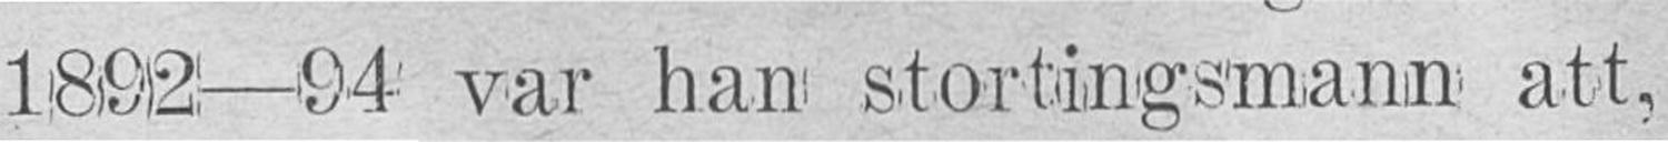1892-94 var han stortingsmann att,
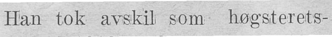han tok avskil som høgsterets-
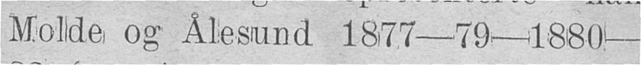Molde og Ålesund 1877-79-1880-
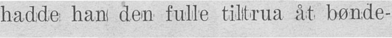hadde han den fulle tiltrua åt bønde.
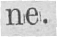ne.
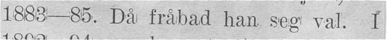1883-85. Då fråbad han seg val. I
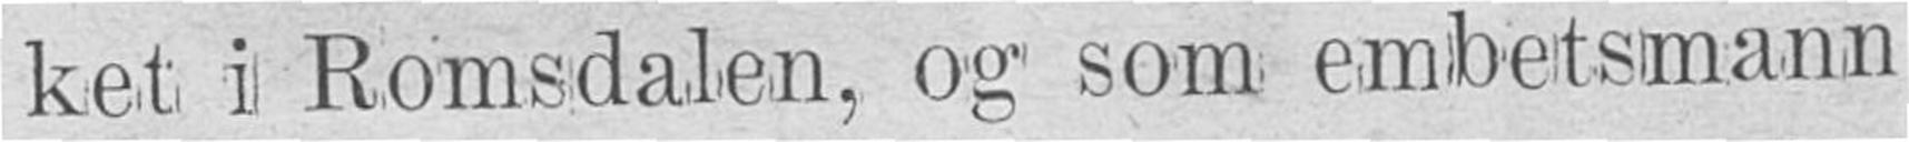ket i Romsdalen, og som embetsmann
